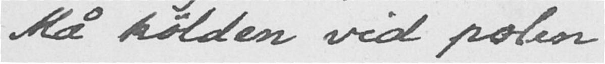Må kälden ved polen
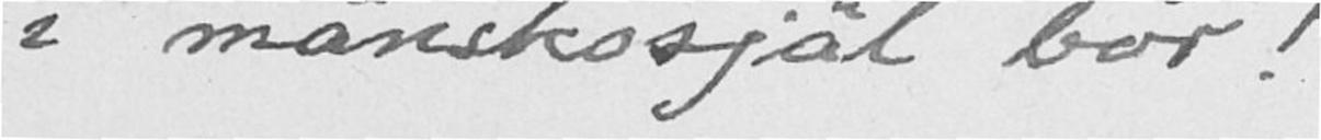i mänskosjät bor!
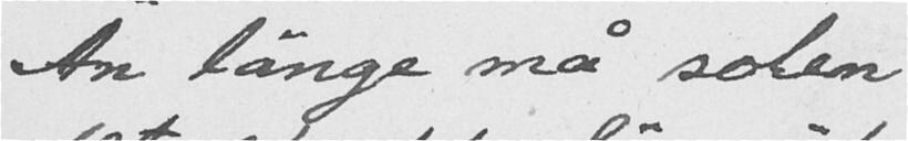An länge må solan
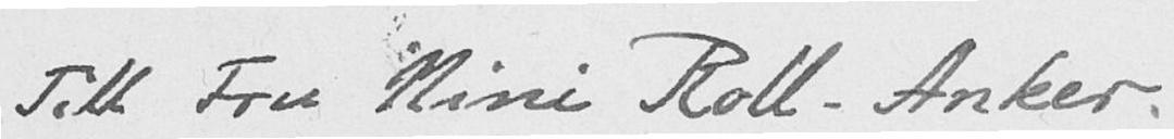Till Fru Nini Roll-Anker.
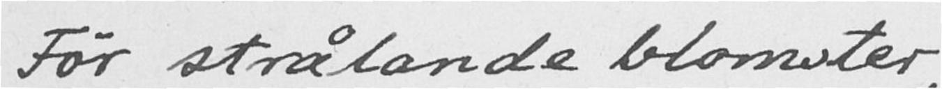För strålande blomster
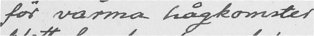för varma hågkonster
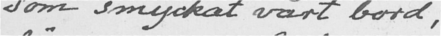som smyckat vårt bord
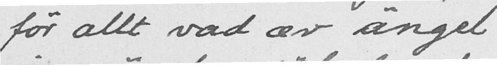för allt vad av ängel
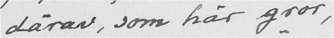därav, som här gror
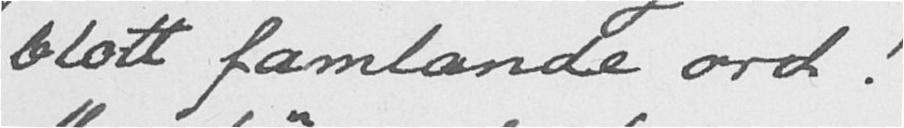blott famlande ord!
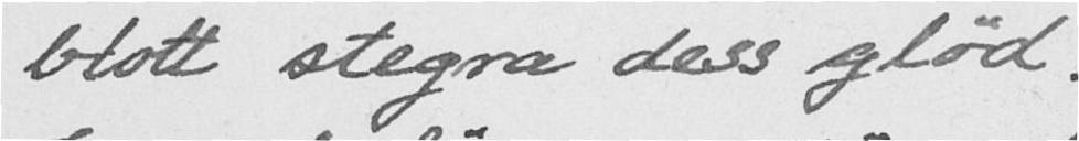blott stegra dess glöd.
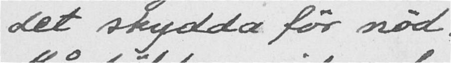det skydda för nöd.
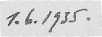1.6.1935.
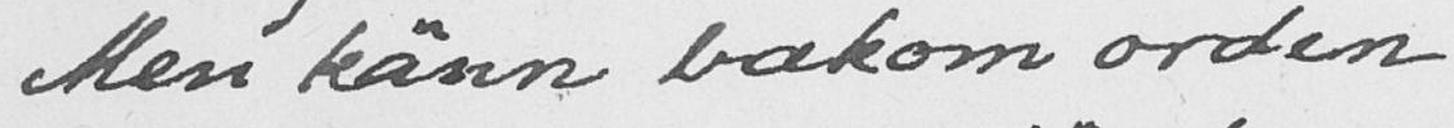Men könn bakom orden
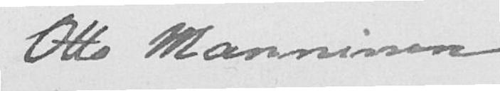Otto Manninn
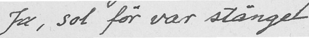Ja, sot för var stänget
